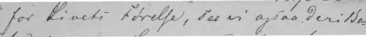for Livets Førelse, see vi ogsaa deri Be-
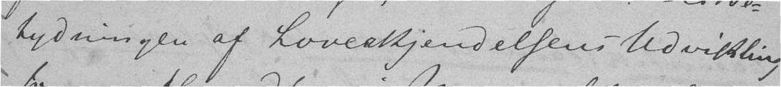tydningen af Loverkjendelsens Udvikling
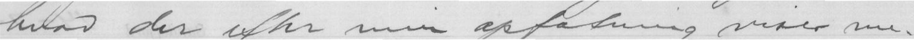hvad der efter min opfatning viser me-
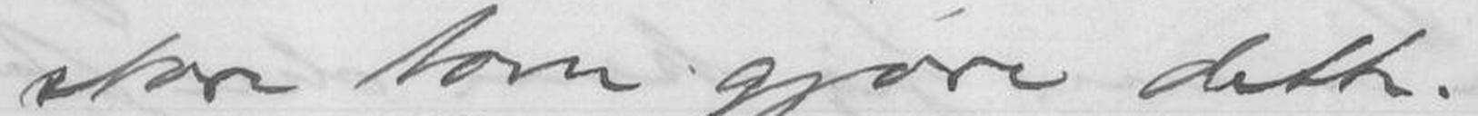store torn gjøre dette.
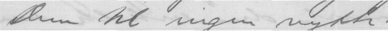Dem til ingen nytte.
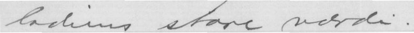lodiens store værdi.
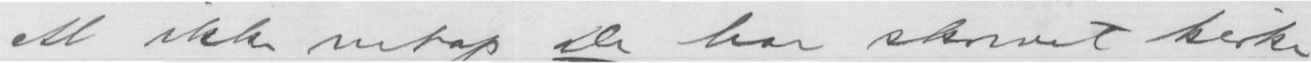At ikke netop De har skrevet kirke
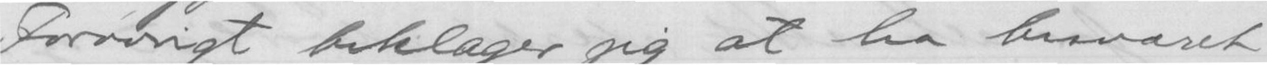Forøvrigt beklager jeg at ha besvaret
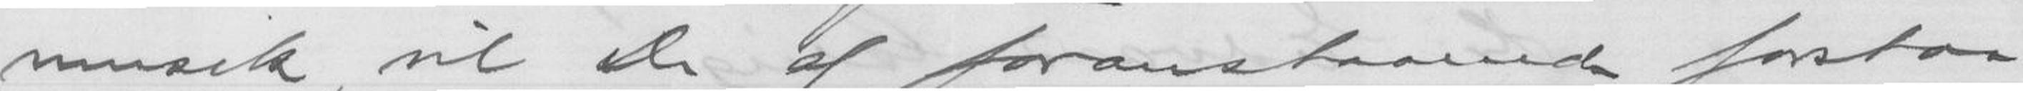musik, vil De af foranstaaende forstaa
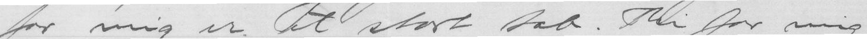for mig er et stort tab. Thi for mig
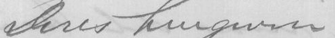Deres Hengivne
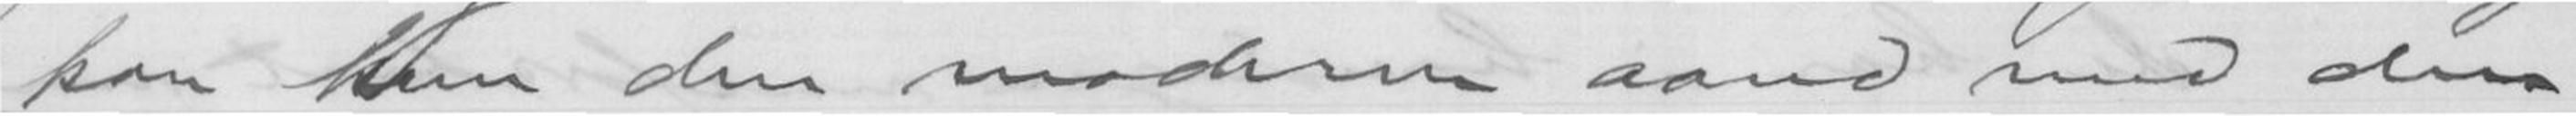kan kun den moderne mand med den
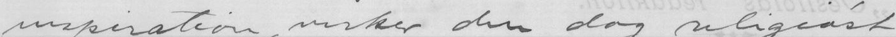inspiration, virker den dog religiøst,
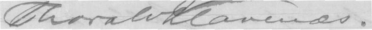Thorvald Klavenæs.
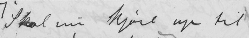Skal nu kjøre op til
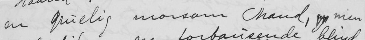en gruelig morsom Mand,  men
